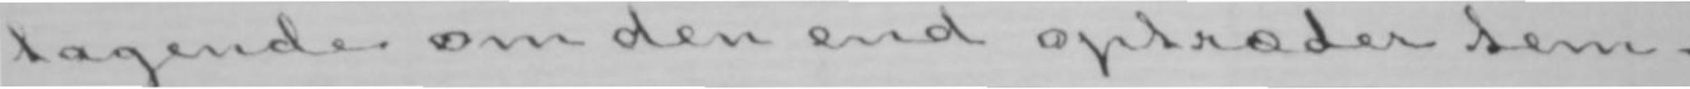tagende om den end optræder tem-
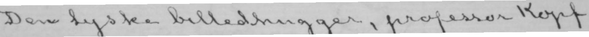Den tyske billedhugger, professor Kopf
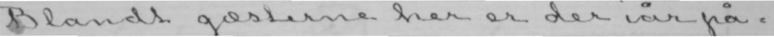Blandt gæsterne her er der iår på-
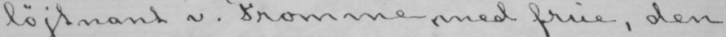løjtnant v. Fromme med frue, den
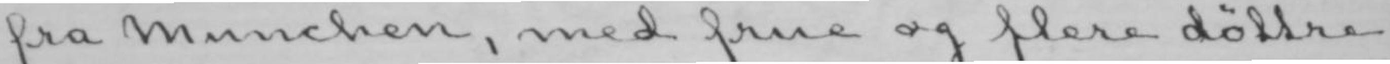fra München, med frue og flere døttre,
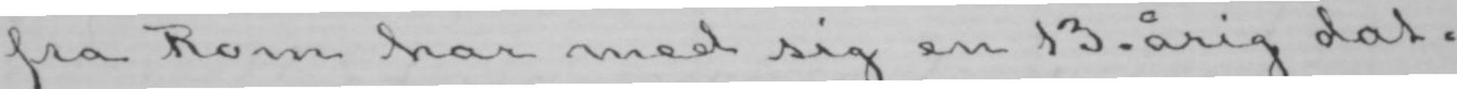fra Rom har med sig en 13-årig dat-
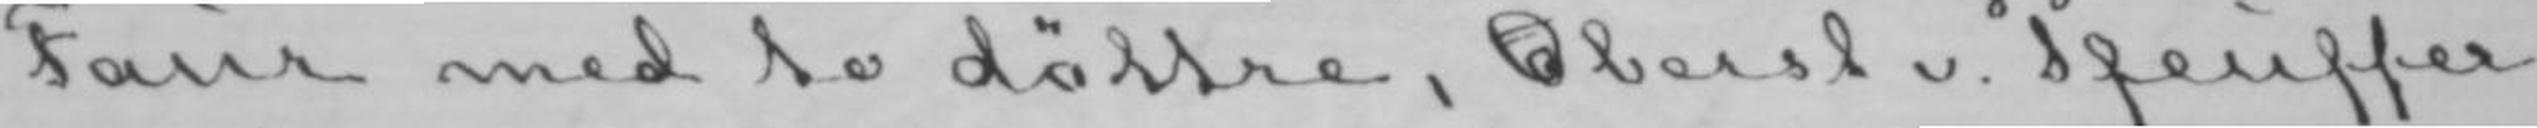Faur med to døttre, Ooberst v. Pfeuffer
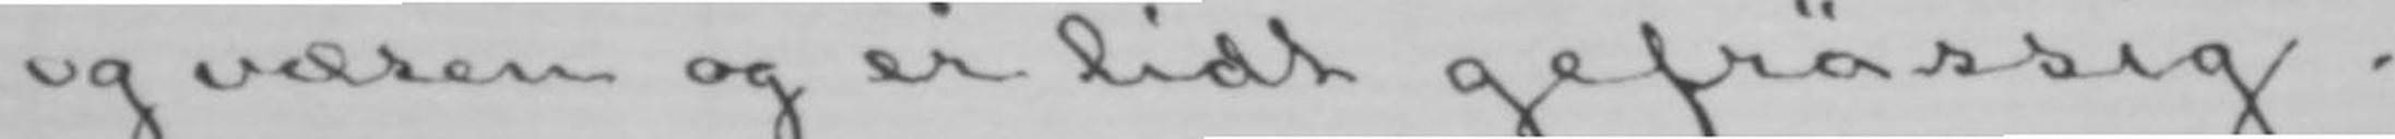og væsen og er lidt gefrässig.
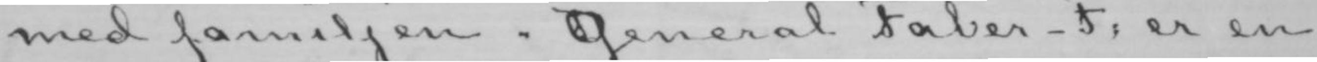med familjen. General Faber-F: er en
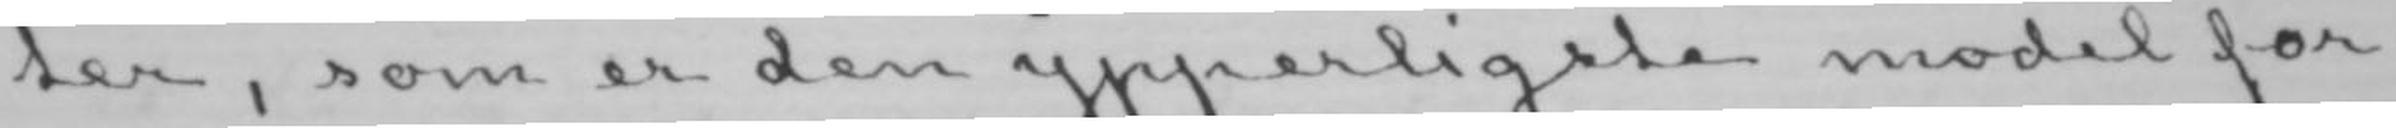ter, som er den ypperligste model for
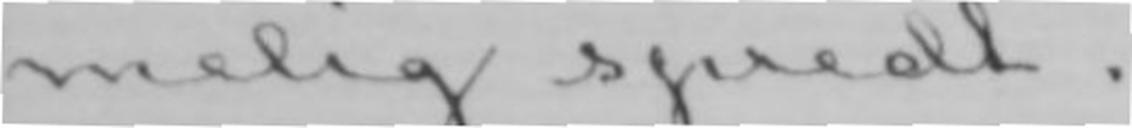melig spredt.
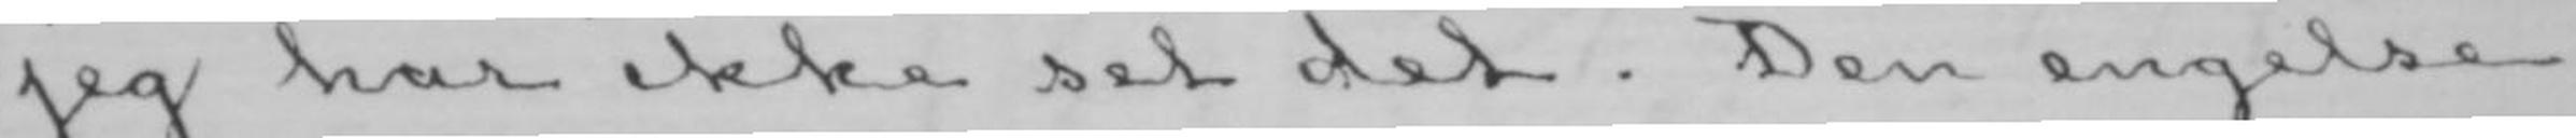jeg har ikke set det. Den engelske
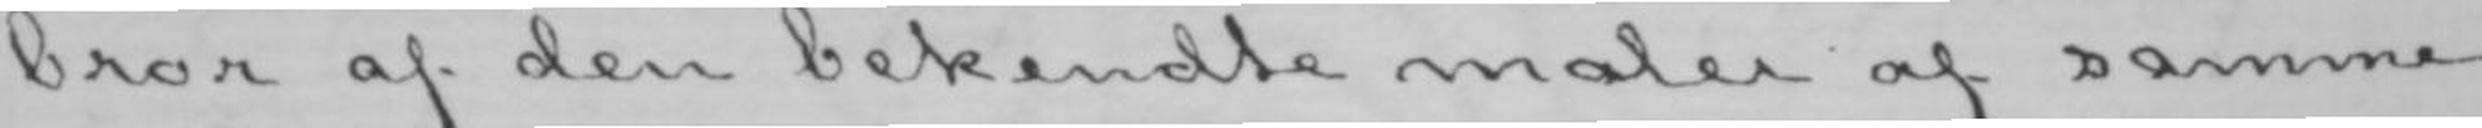bror af den bekendte maler af samme
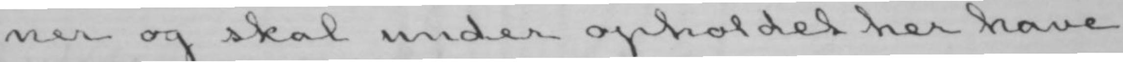ner og skal under opholdet her have
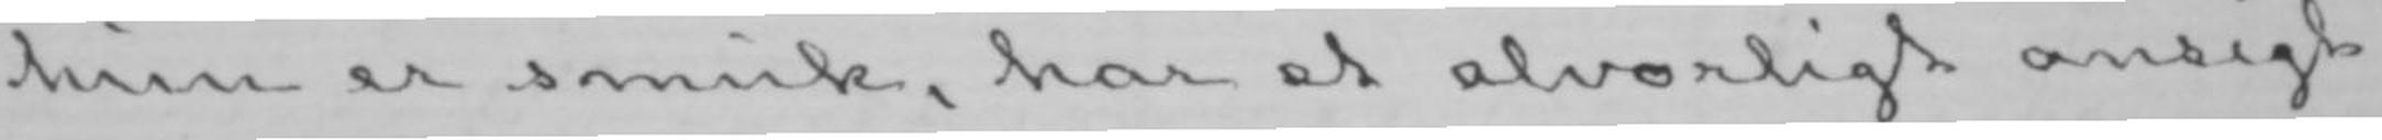hun er smuk, har et alvorligt ansigt
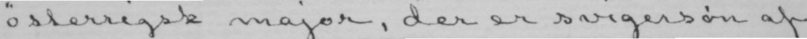østerrigsk major, der er svigersøn af
